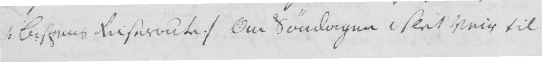/: Bispens Reiserude :/ Om Søndagen i slet Veir til
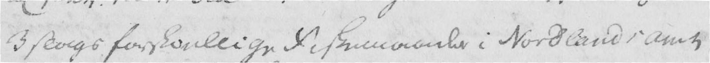3 slags forskiellige Fiskemaader i Nordlands Amt
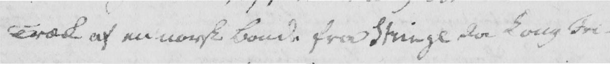Træk af en norsk Bonde fra Stringe da Kong Fre-
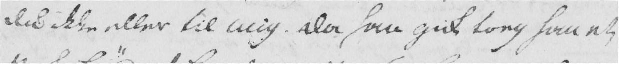du icke eller til mig, da han gik toeg han et
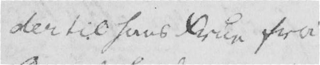der til hans Frue fra
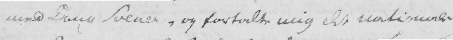med Kong Sverre, og fortalte mig det nationale
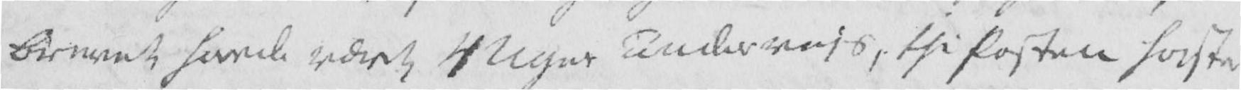Brevet havde været 4 Uger undervejs, thi Posten haste
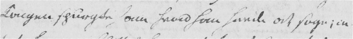Kongen spurgte ham hvad han havde at sige; in-
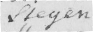Stegen
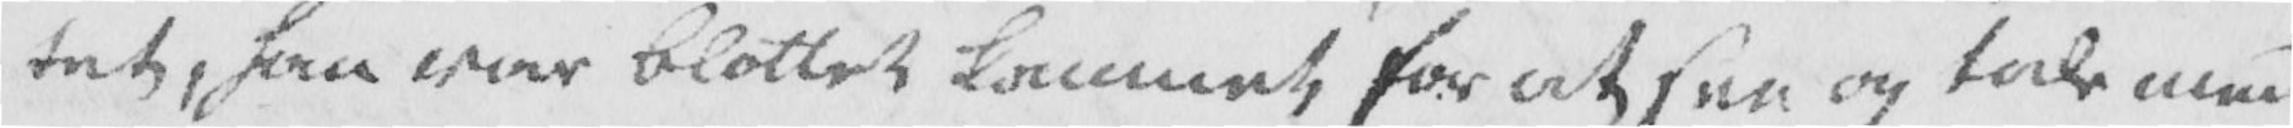tet, han var blottet kommet for at see og tale med
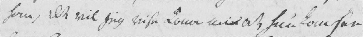han, Det vil jeg vise Kona mi at hun kan see
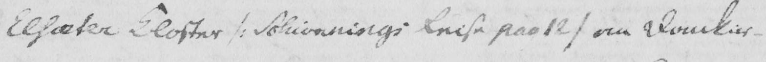Elsæter Kloster /: Schionnings Reise paa 12 :/ om Domkir-
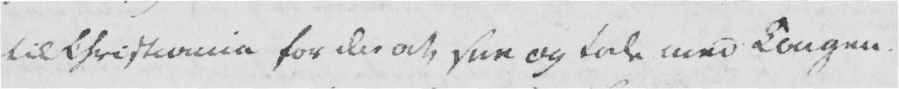til Christiania for der at see og tale med Kongen.
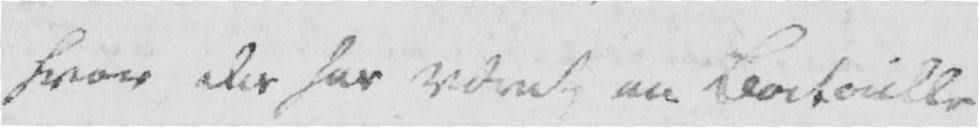hvor der har været en Bataille
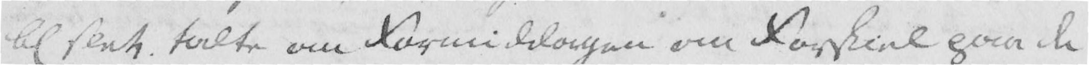bl slet. talte om Formiddagen om Forskiel paa de
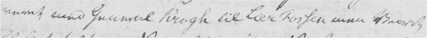været med General Krogh til Lier-Fossen men Veiret
